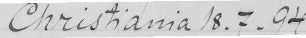Christiania 18.7.94
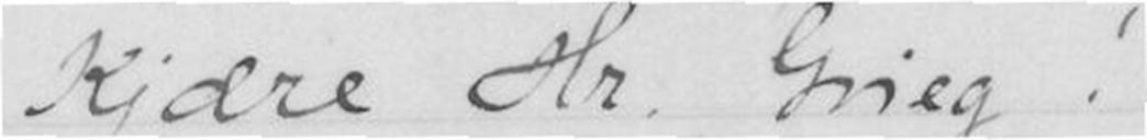Kjære Hr. Grieg!
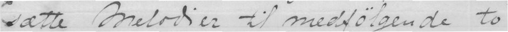sætte Melodier til medfølgende to
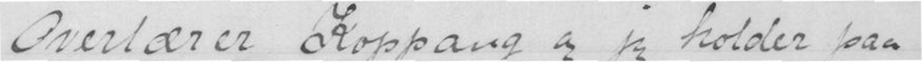Overlærer Koppang og jeg holder paa
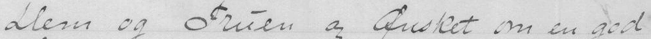Dem og Fruen og Ønsket om en god
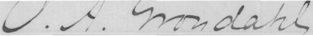O.A. Grøndahl
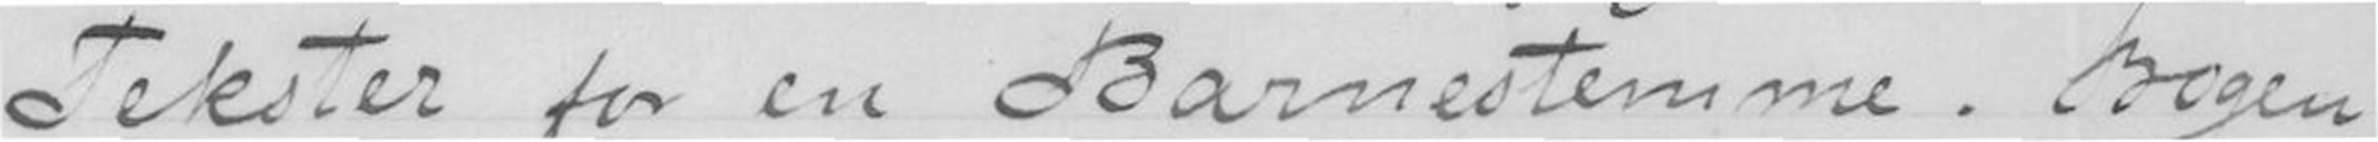Tekster for en Barnestemme. Bogen
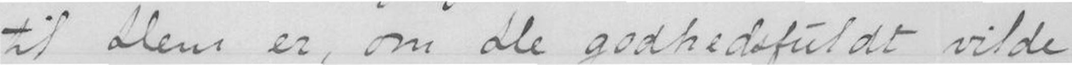til Dem er, om De godhedsfuldt vilde
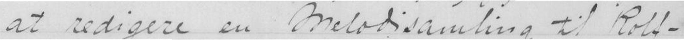at redigere en Melodisamling til Rolf-
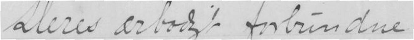Deres ærbøge forbundne
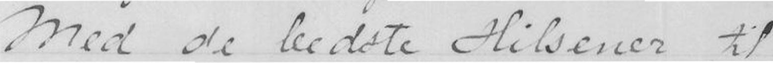Med de bedste Hilsener til
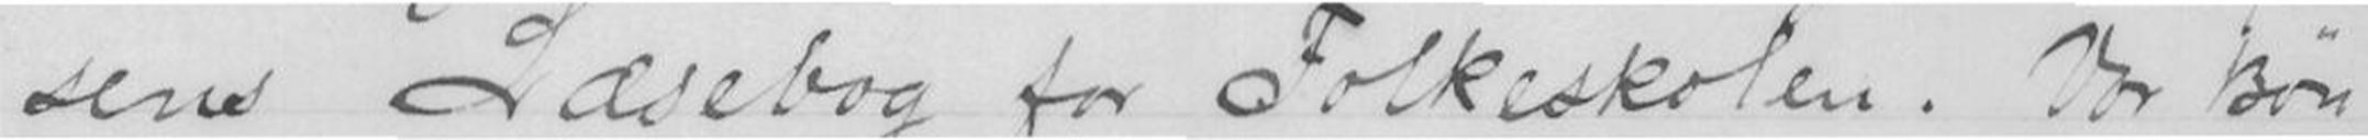sens Lærerbog for Folkeskolen. Vor Bøn
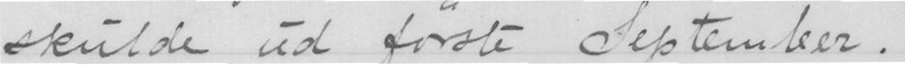skulde ud første September.
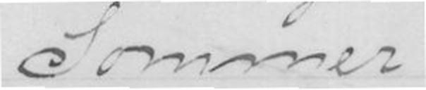sommer.
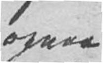opnaa
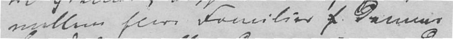mellem flere Familier f danner Stemme til (paa),
